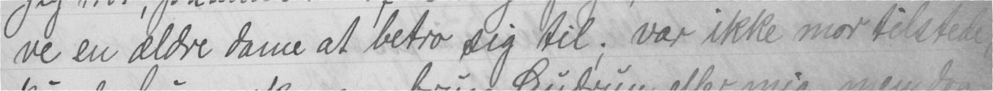ve en ældre dame at betro sig til; var ikke mor tilstede,
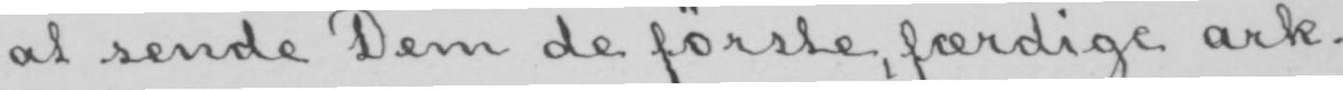at sende Dem de første, færdige ark.
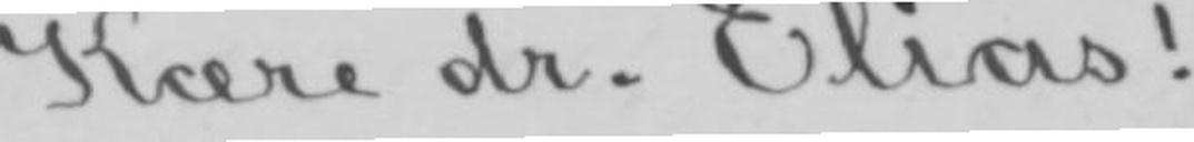Kære dr. Elias!
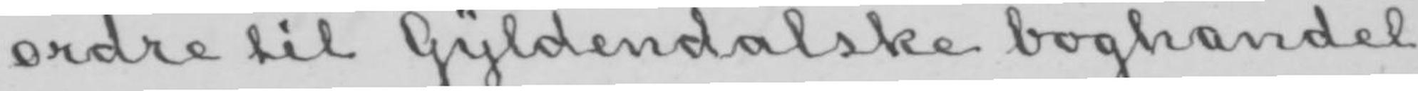ordre til Gyldendalske boghandel
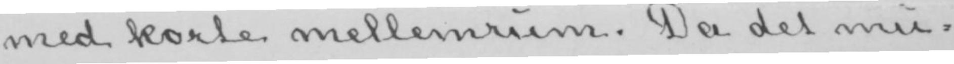med korte mellemrum. Da det mu-
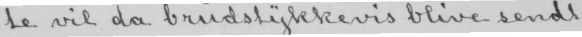ter vil da brudstykkevis blive sendt
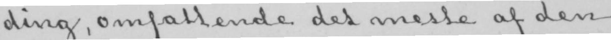ding, omfattende det meste af den
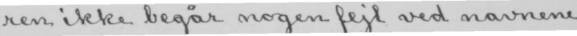ren ikke begår nogen fejl ved navnene
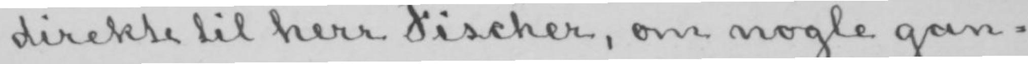direkte til herr Fischer, om nogle gan-
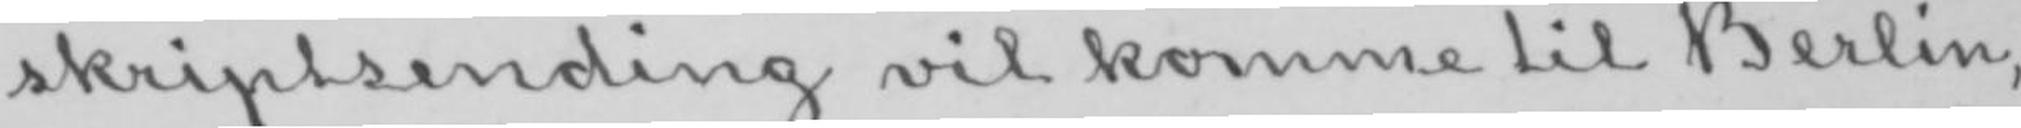skriptsending vil komme til Berlin,
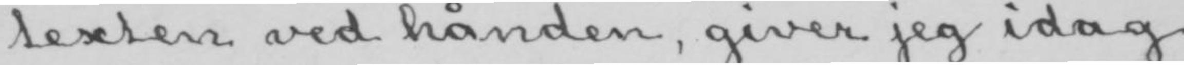texten ved hånden, giver jeg idag
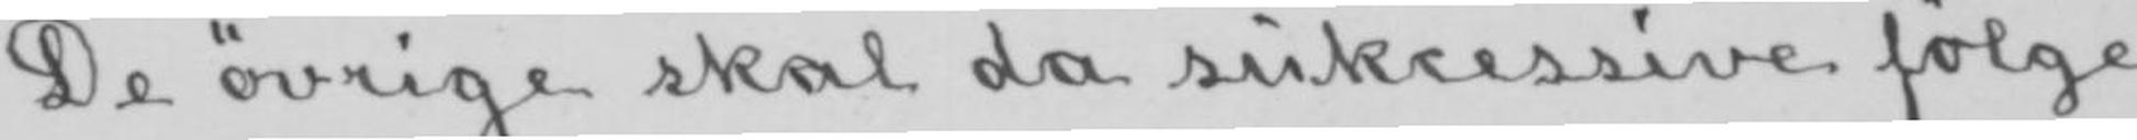De øvrige skal da sukcessive følge
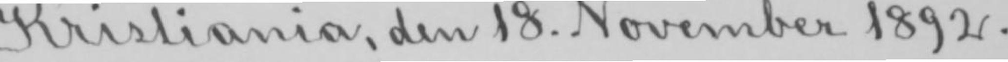Kristiania, den 18. November 1892.
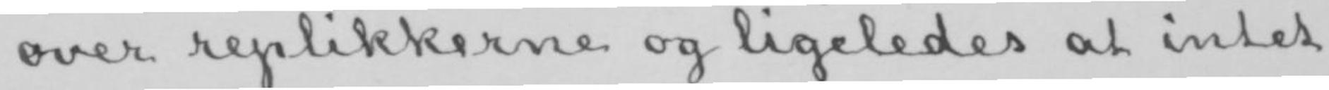over replikkerne og ligeledes at intet
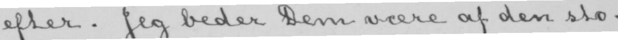efter. Jeg beder Dem være af den sto-
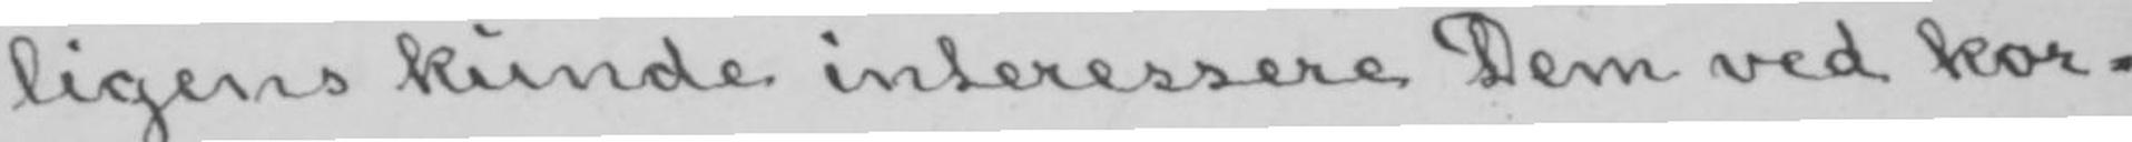ligens kunde interessere Dem ved kor-
